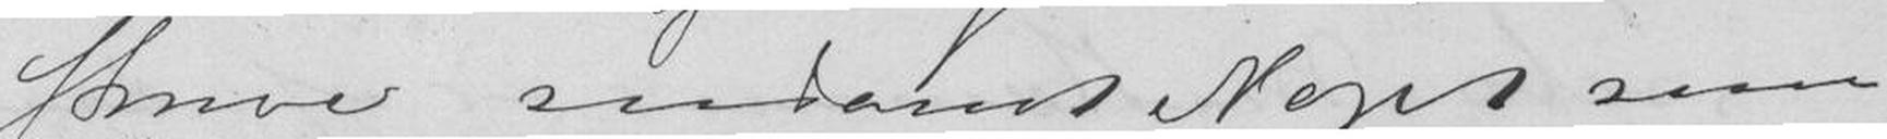skrive saadant Noget som
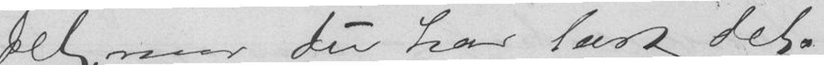Det naar du har læst det.
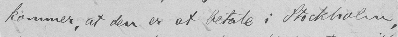kommer, at den er at betale i Stockholm,
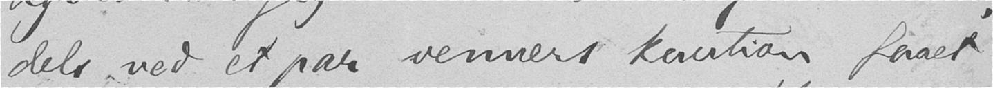dels ved et par venners kaution faaet
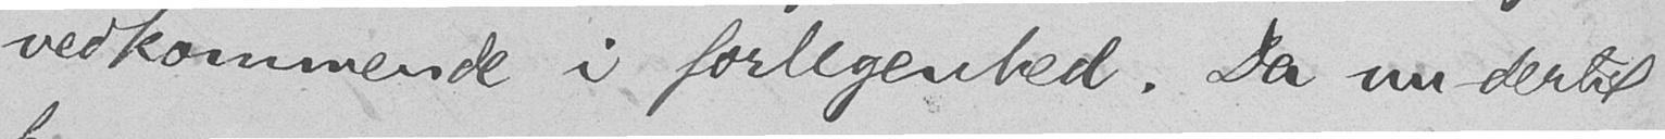vedkommende i forlegenhed. Da nu dertil
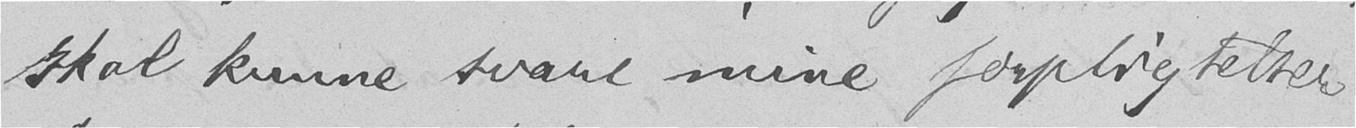skal kunne svare mine forpligtelser.
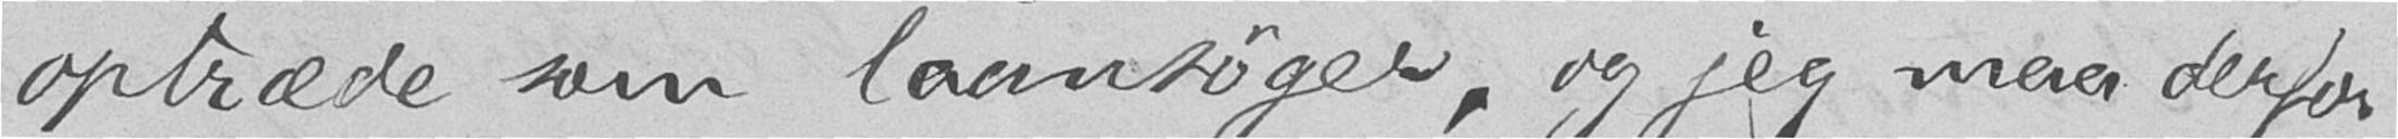optræde som laansøger, og jeg maa derfor
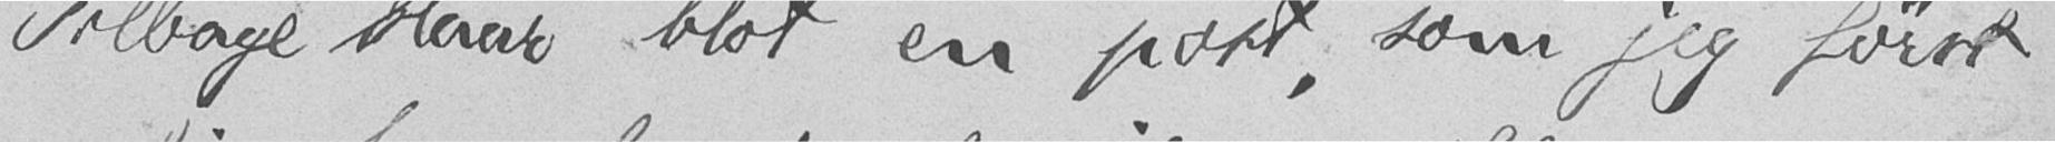Tilbage staar blot en post, som jeg først
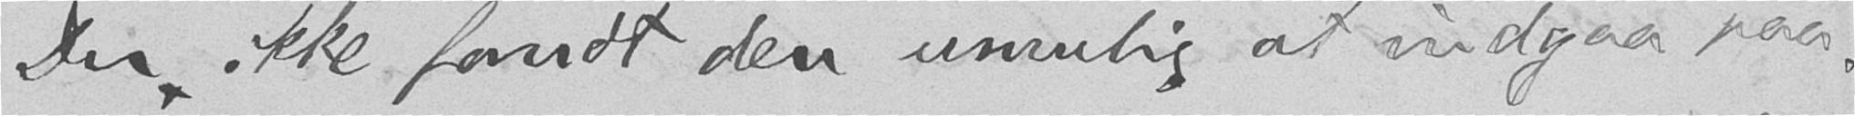Du ikke fandt den umulig at indgaa paa.
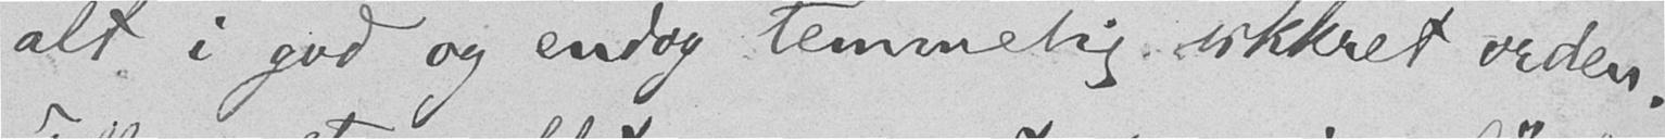alt i god og endog temmelig sikkret orden.
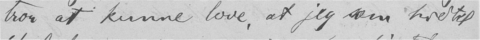tror at kunne love, at jeg som hidtil
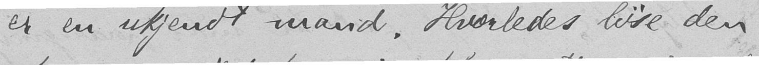er en ukjendt mand. Hvorledes løse den
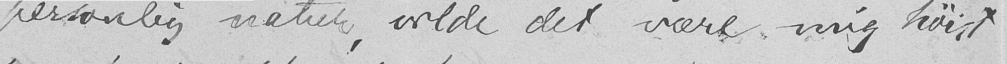personlig natur, vilde det være mig høist
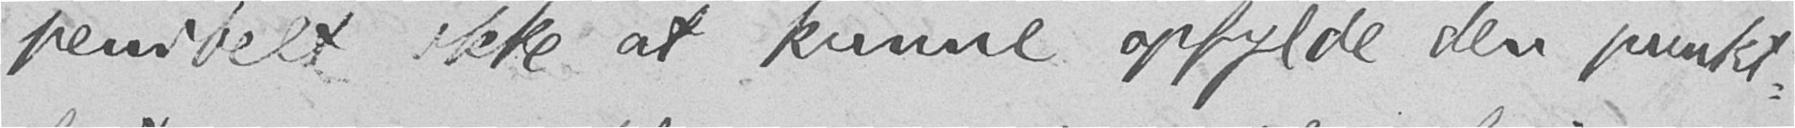penibelt ikke at kunne opfylde den punkt-
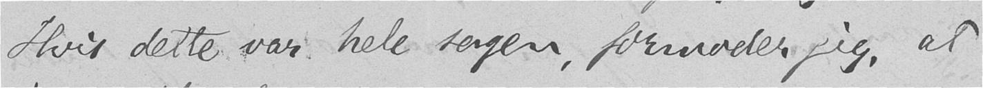Hvis dette var hele sagen, formoder jeg, at
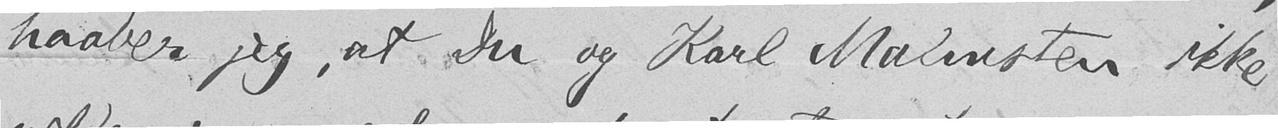haaber jeg, at Du og Karl Malmsten ikke
